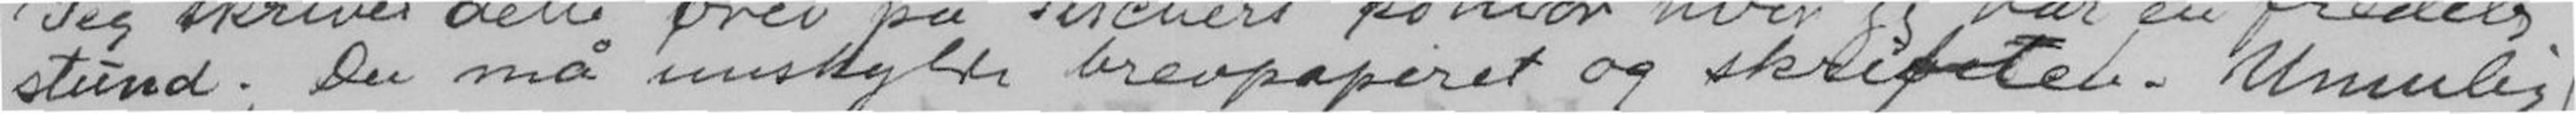stund. Du må unskylde brevpapiret og skriften. Umulig
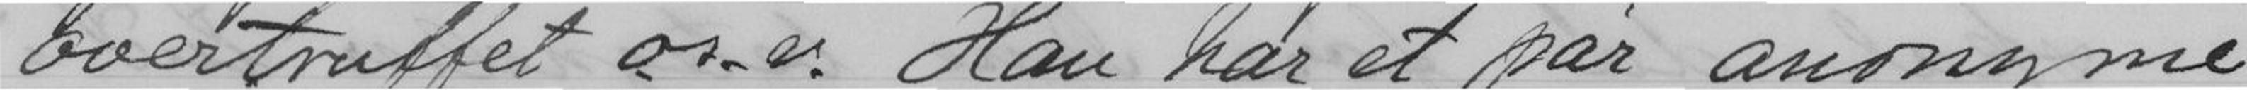overtruffet o.s.v. Han har et par anonyme
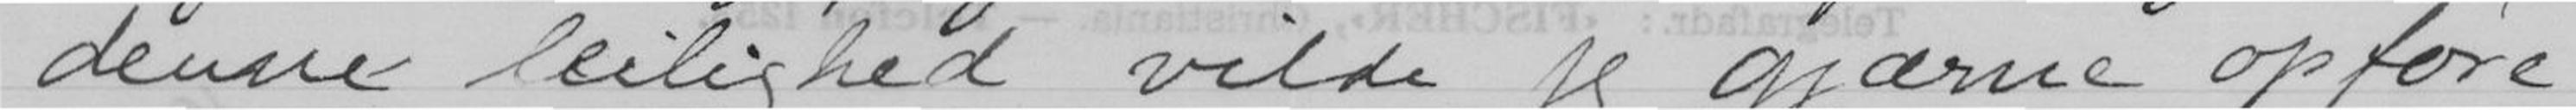denne leilighed vilde jeg gjærne opføre
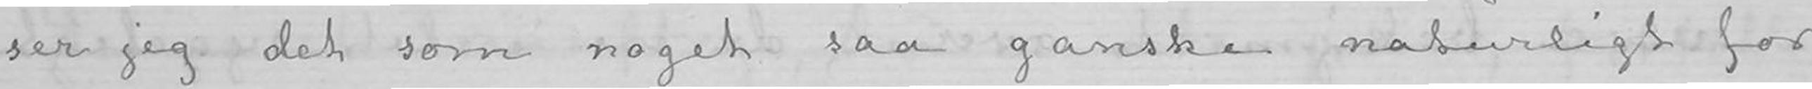ser jeg det som noget saa ganske naturligt for
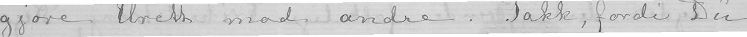gjøre Urett mod andre. Takk, fordi Du
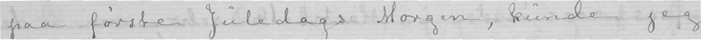paa første Juledags Morgen, kunde jeg
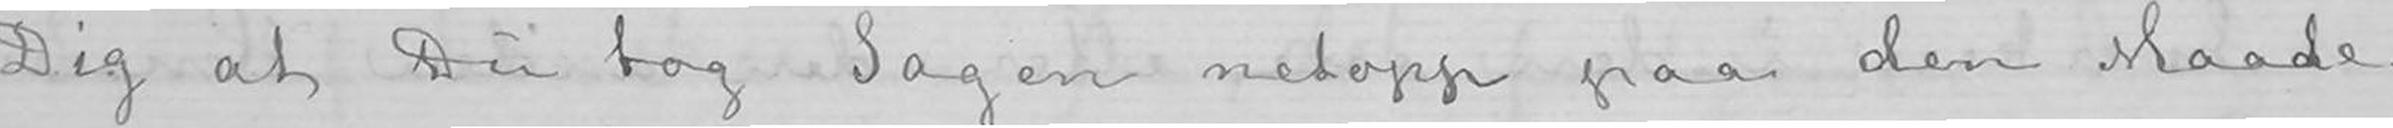Dig at Du tog Sagen netopp paa den Maade
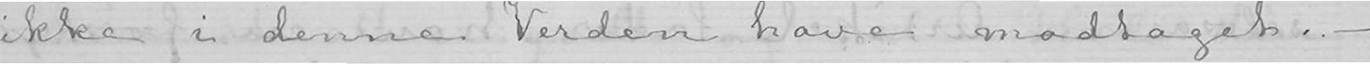ikke i denne Verden have modtaget.-
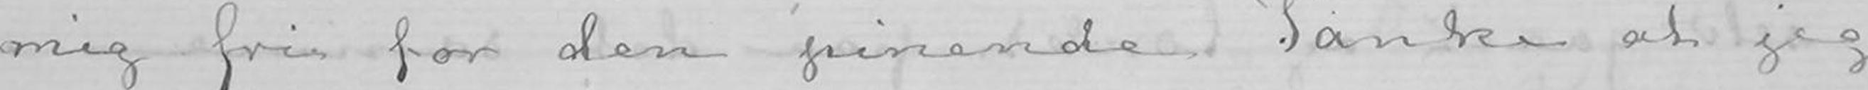mig fri for den pinende Tanke at jeg
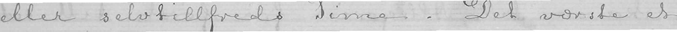eller selvtillfreds Time. Det værste et
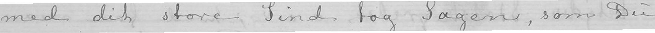med dit store Sind tog Sagen, som Du
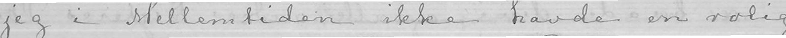jeg i Mellemtiden ikke havde en rolig
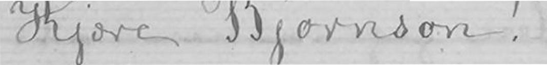Kjære Bjørnson!
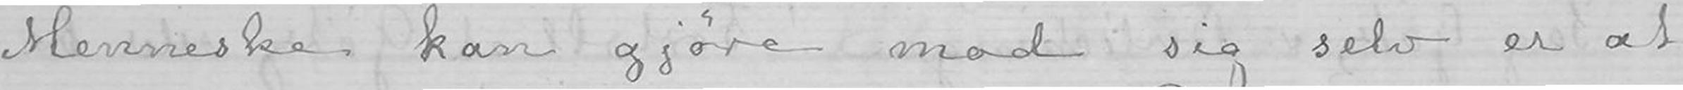Menneske kan gjøre mod sig selv er at
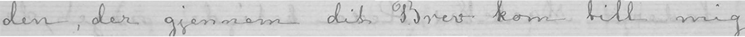den, der gjennem dit Brev kom till mig
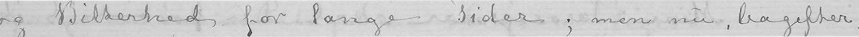og Bitterhed for lange Tider; men nu, bagefter,
